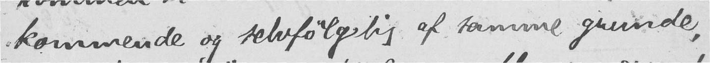kommende og selvfølgelig af samme grunde,
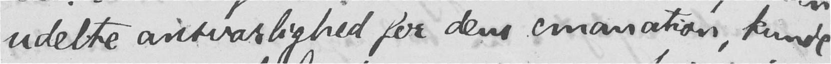udelte ansvarlighed for dens emanation, kunde
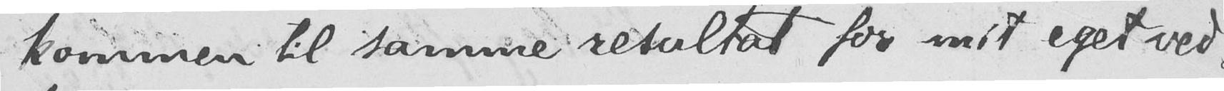kommen til samme resultat for mit eget ved-
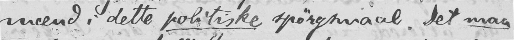mænd i dette politiske spørgsmaal. Det maa
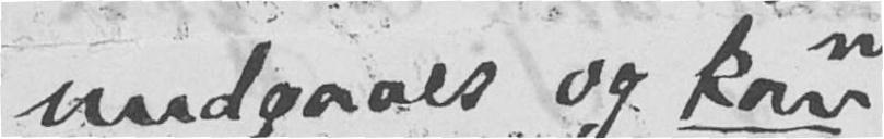undgaaes og kan
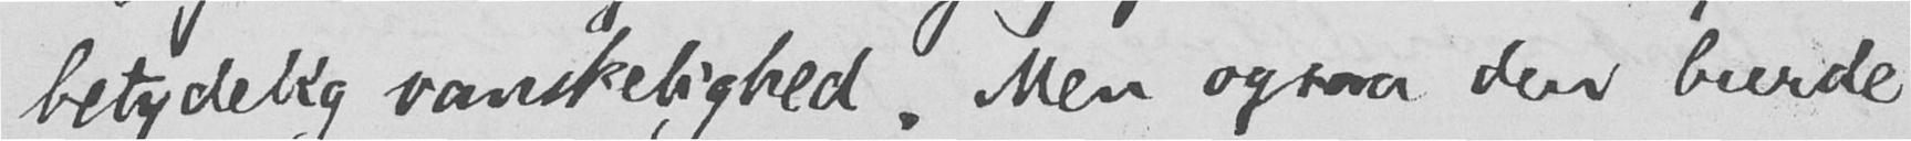betydelig vanskelighed. Men ogsaa den burde
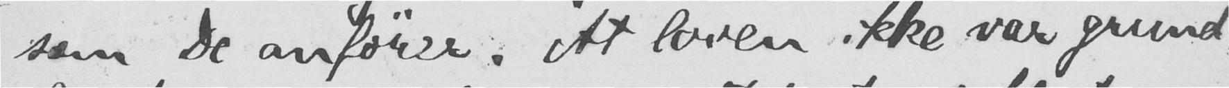som De anfører. At loven ikke var grund-
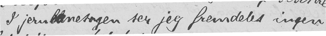I jernbanesagen ser jeg fremdeles ingen
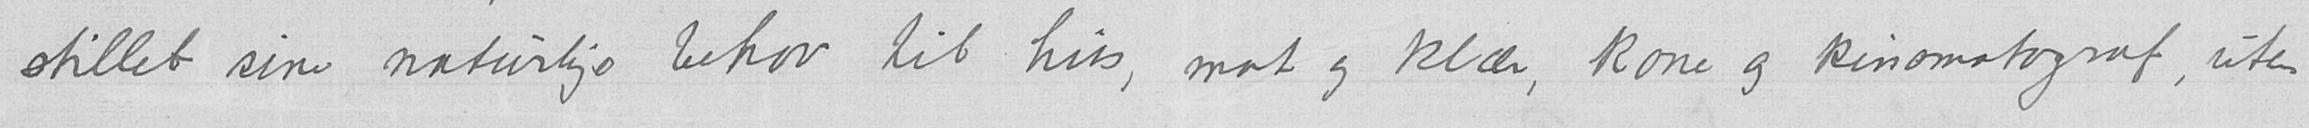stillet sine naturlige behov til hus, mat og klær, kone og kinomatograf, uten
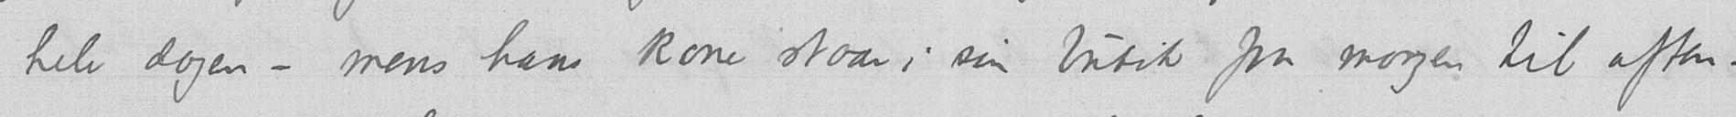hele dagen – mens hans kone staar i sin butik fra morgen til aften.
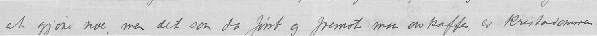at gjøre noe, men det som da først og fremst maa avskaffes, er kristendommen.
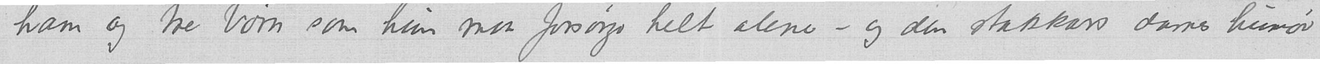ham og tre børn som hun maa forsørge helt alene – og den stakkars dames humør
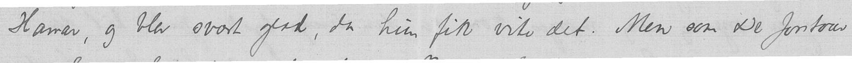Hamar, og blev svært glad, da hun fik vite det. Men som De forstaar
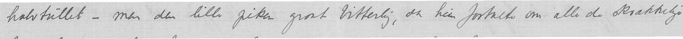halvtullet – men den lille piken graat bitterlig, da hun fortalte om alle de skrækkelige
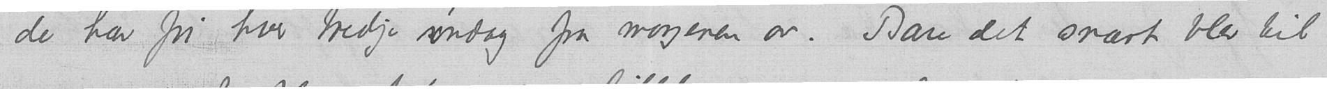de har fri hver tredje søndag fra morgenen av. Bare det snart blev til
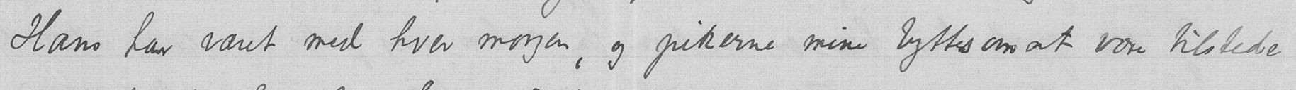Hans har været med hver morgen, og pikerne mine byttes om at være tilstede
